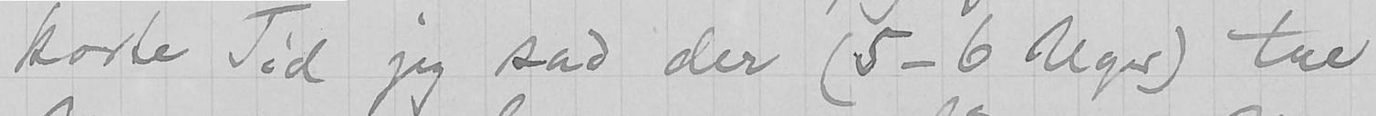korte Tid jeg sad der (5-6 Uger) tre
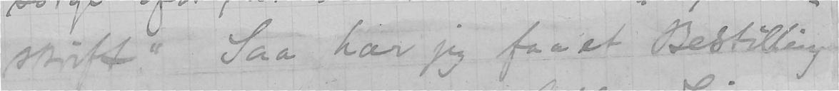skrift." Saa har jeg faaet Bestilling
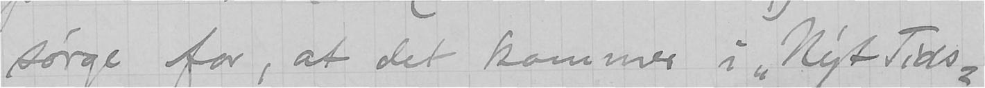sørge for, at det kommer i "Nyt Tids-
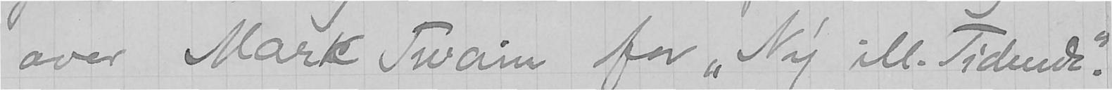over Mark Twain for "Ny ill. Tidende".
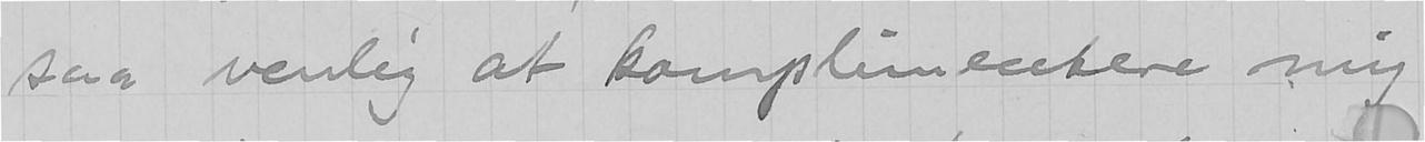saa venlig at komplimentere mig
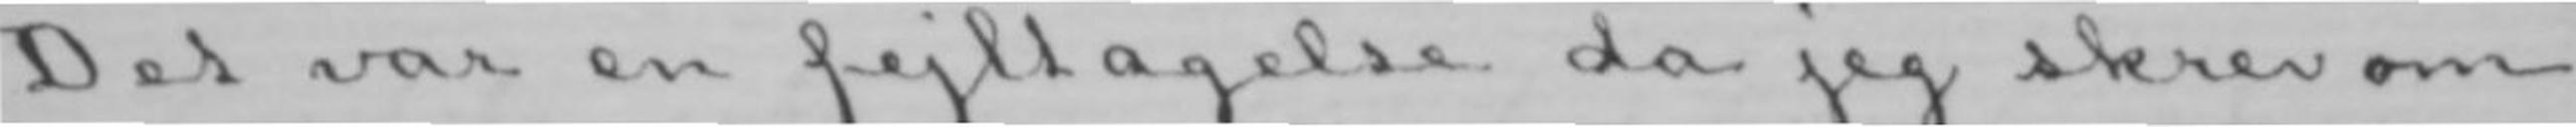Det var en fejltagelse da jeg skrev om
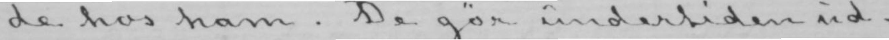de hos ham. De gør undertiden ud-
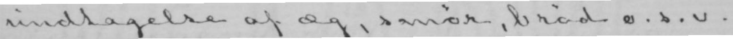undtagelse af æg, smør, brød o. s. v.
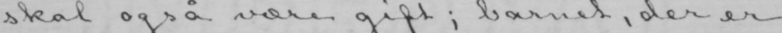skal også være gift; barnet, der er
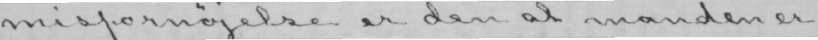misfornøjelse er den at manden er
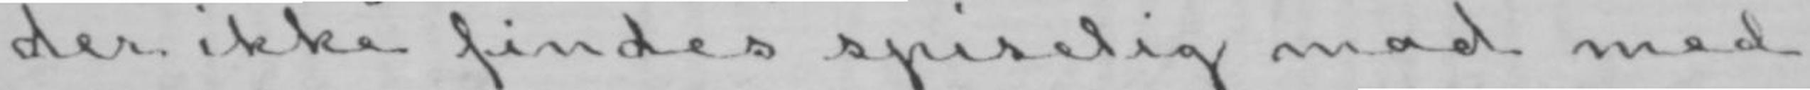der ikke findes spiselig mad med
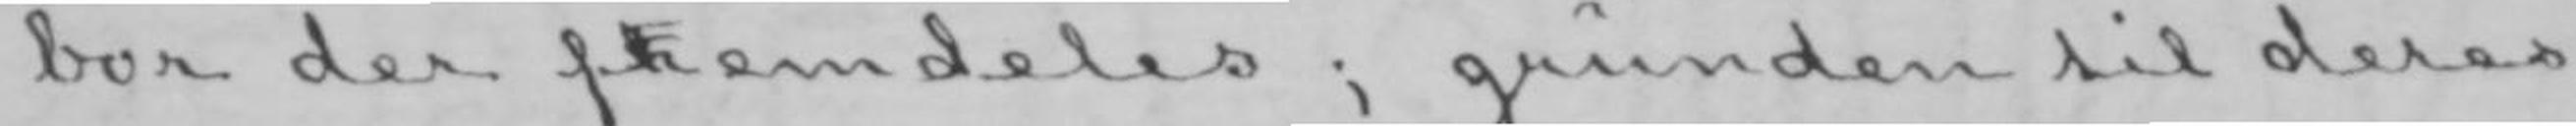bor der fremdeles; grunden til deres
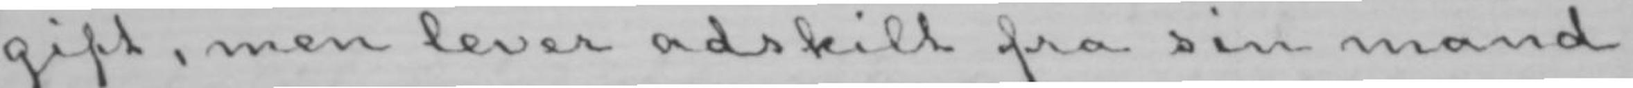gift, men lever adskilt fra sin mand,
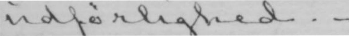udførlighed.-
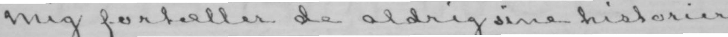Mig fortæller de aldrig sine historier
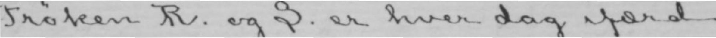Frøken R. og S. er hver dag ifærd
